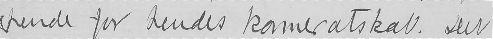hende for hendes kameratskab. Det
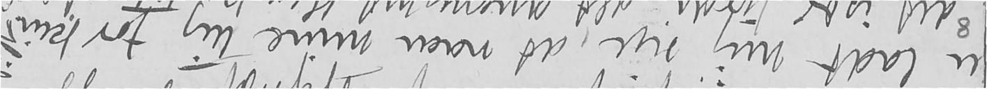u ladt mig sige, at naar mine ting for kun
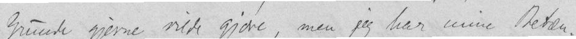Grunde gjerne vilde gjøre, men jeg har mine Betæn-
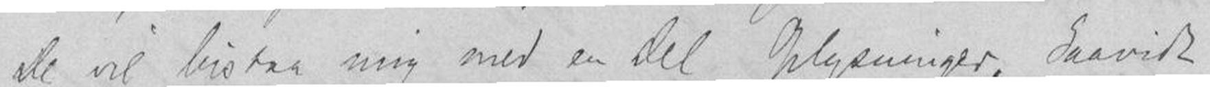de vil bistaa mig med en del Oplysninger. Saavidt
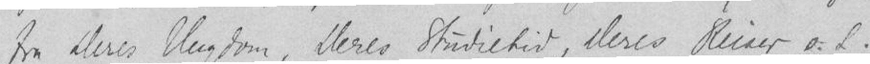fra Deres Ungdom, Deres Studietid, Deres Reiser o.l.
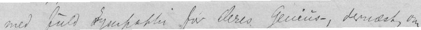med fuld Synpathi for deres Genius-, dernæst om
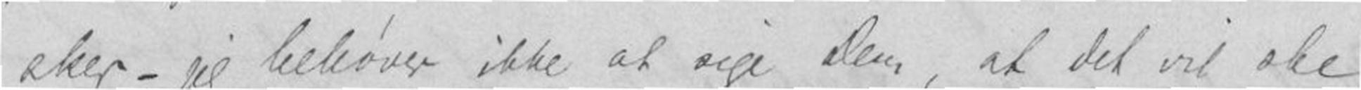sker - jeg behøver ikke at sige dem, at det vil øke
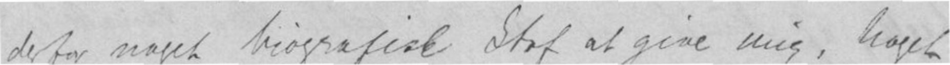derfor noget biografisk Stof at give mig, noget
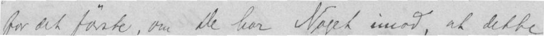for det første, om De har Noget imod, at dette
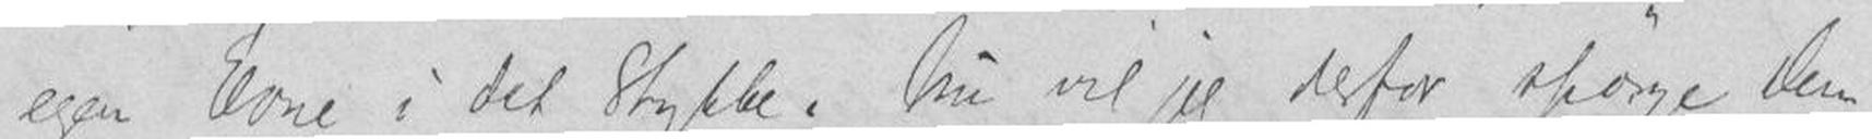egen Evne i det Stykke. Nu vil jeg derfor spørge dem
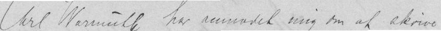Carl Warmuth har anmodet mig om at skrive
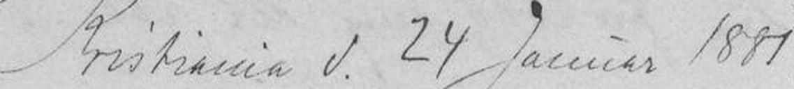Kristiania d.24 Januar 1881
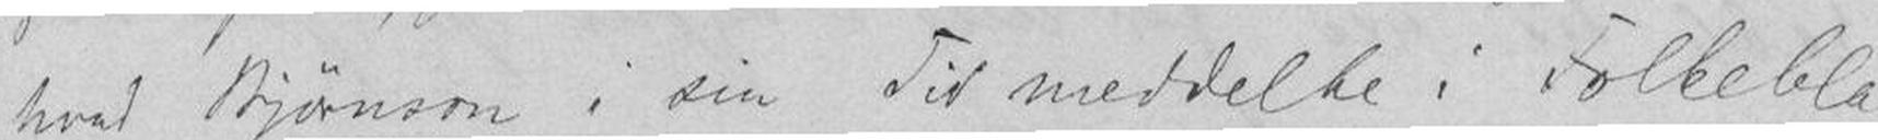hva Bjørnson i sin Tid meddelte i Folkebla-
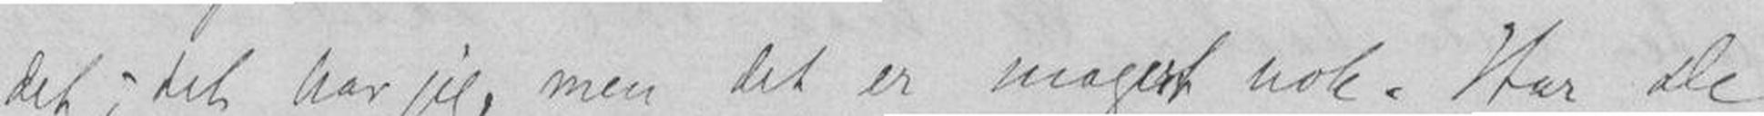det; det har jeg, men det er magert nok. Har de
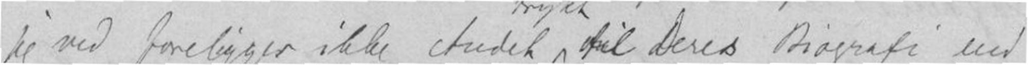jeg ved foreligger ikke Andet til Deres Biografi end
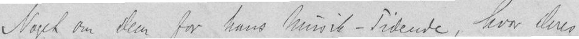Noget om den for hans Musik - Tidende, hvor Deres
